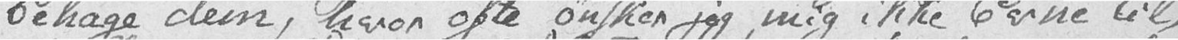behage dem, hvor ofte ønsker jeg mig ikke Evne til,
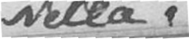Nella.
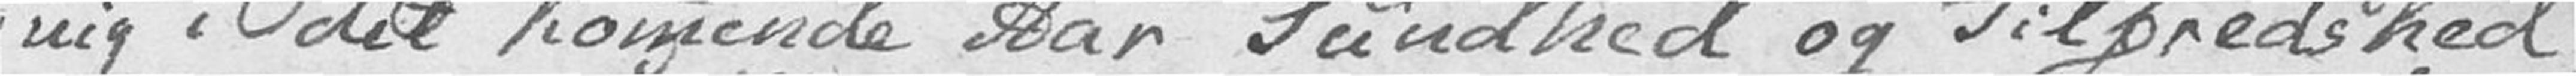mig i det kommende Aar Sundhed og Tilfredshed
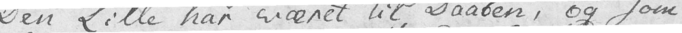Den Lille har været til Daaben, og som
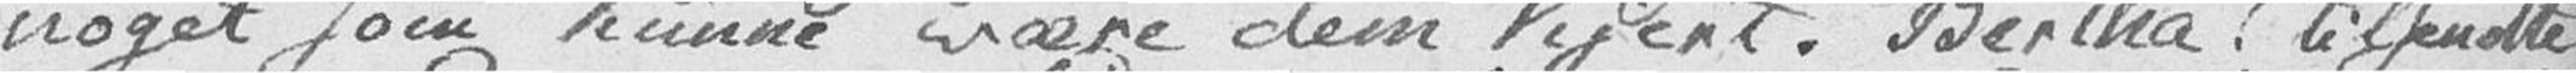noget som kunne være dem kjent. Bertha! tilsendte
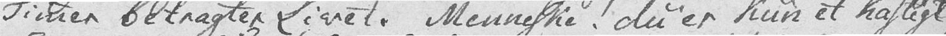Timmer betragter Livet. Menneske! du er kun et hastigt
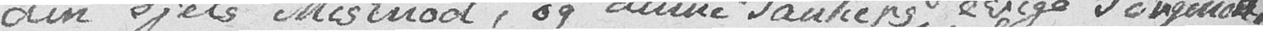din Sjels Mismod, og dinne Tankers evige Sørgmod.
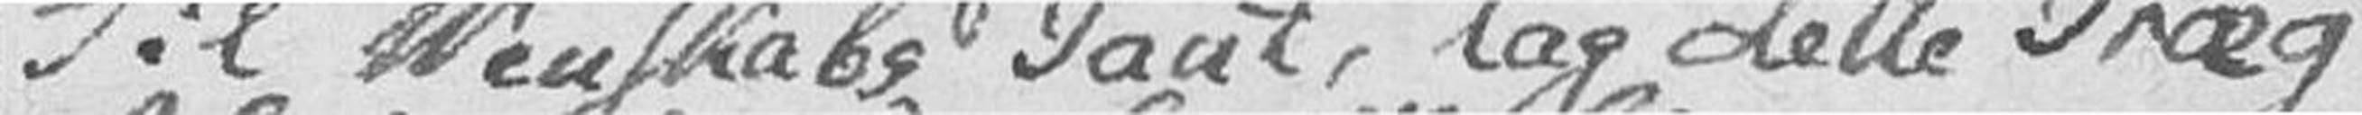Til Venskabs Pant, tag dette Præg
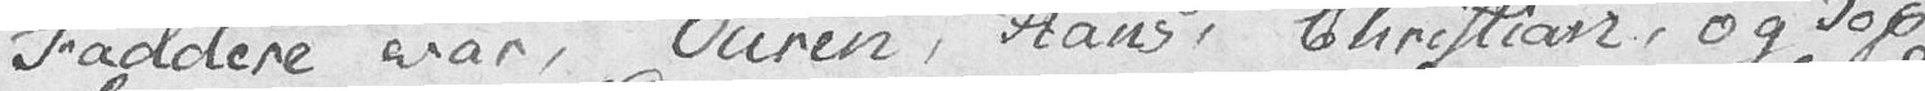Faddere var, Ouren, Hans, Christian, og Sop
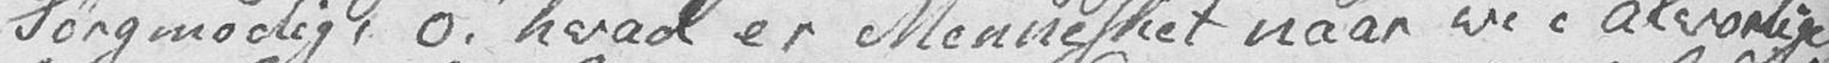Sørgmodig, o! hvad er Mennesket naar vi i alvorlige
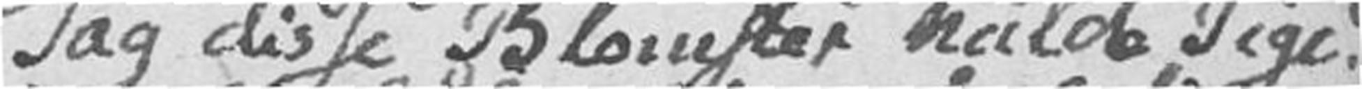Tag disse Blomster Hulde Pige!
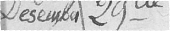Desember 29de
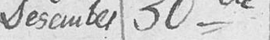Desember 30de
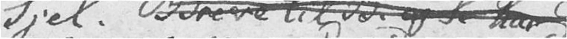Sjel. Breve til B og S har j
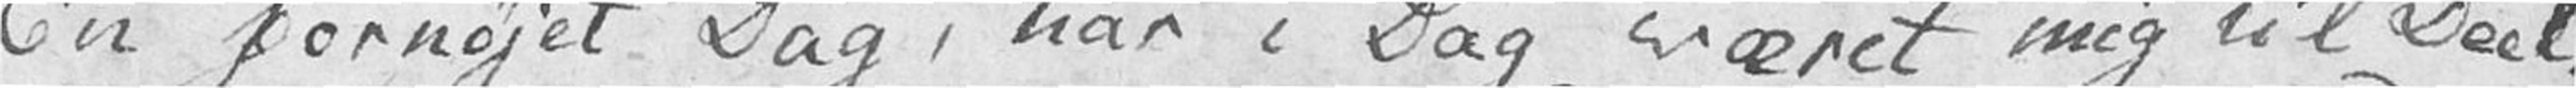En fornøjet Dag, har i Dag været mig til Deel.
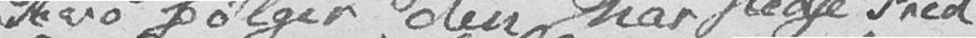Hvo følger den har stedse Fred
